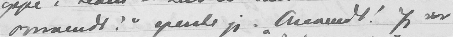anvendt! speiste jeg. "Anvendt!" Jeg var
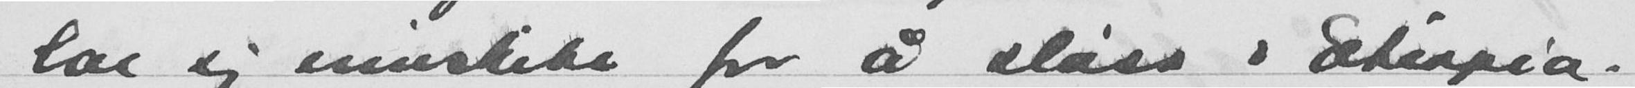lar sig mislike for å slåss i Etiopia.
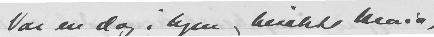Var en dag i byen og besøkte Maia,
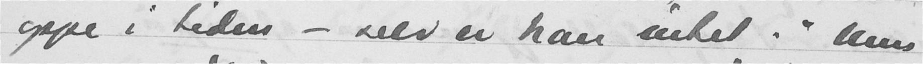oppe i tiden - selv er han intet. Kun
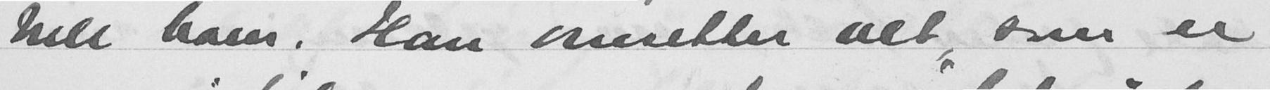hele ham. Han omsetter alt, som er
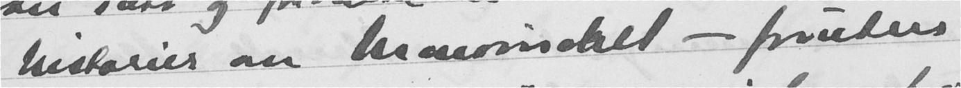historier om Mowinckel - foruten
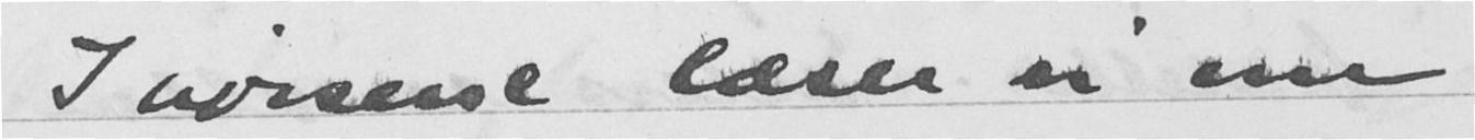I avisene læser vi om
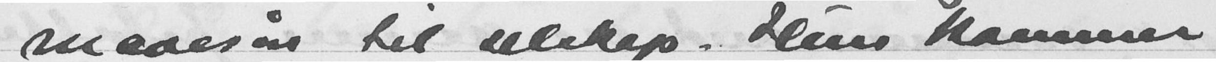mavesår til selskap. Hun kommer
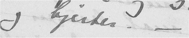og hjerter. -
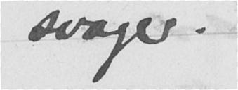svoger.
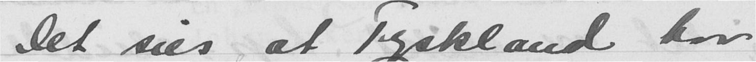Det sies, at Tyskland har
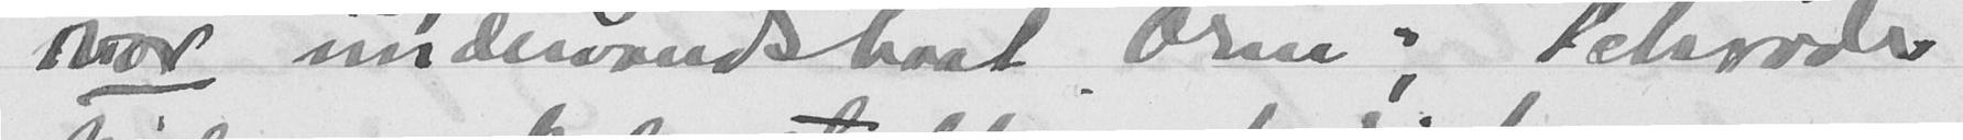vor undervandsbaat "Orm", Schrøder
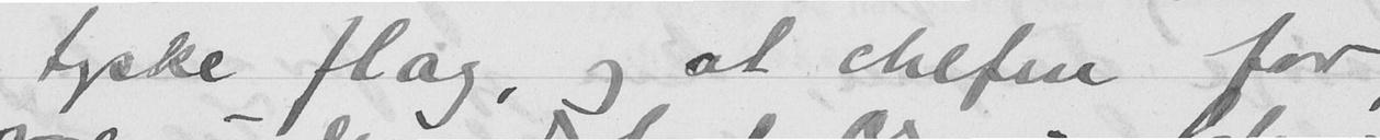tyske flag, og at chefen for
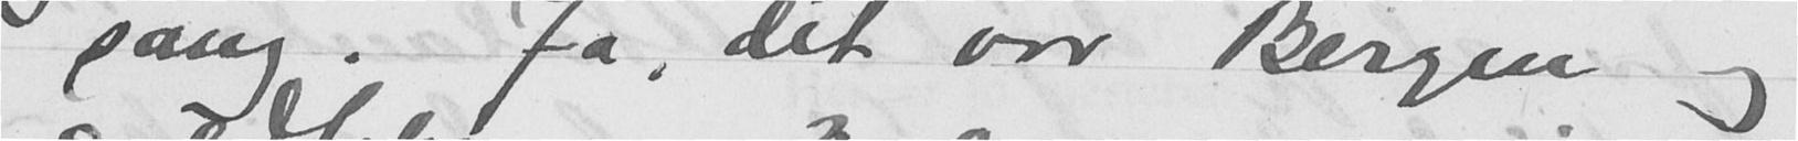sang. Ja, det var Bergen og
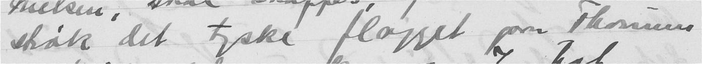strøk det tyske flagget paa Thorum
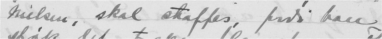Nielsen, skal straffes, fordi han
